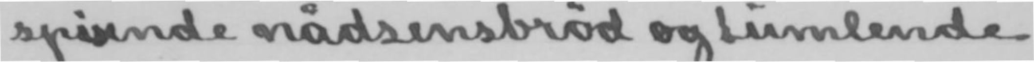spisende nådsensbrød og tumlende
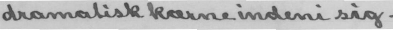dramatisk kærne indeni sig.
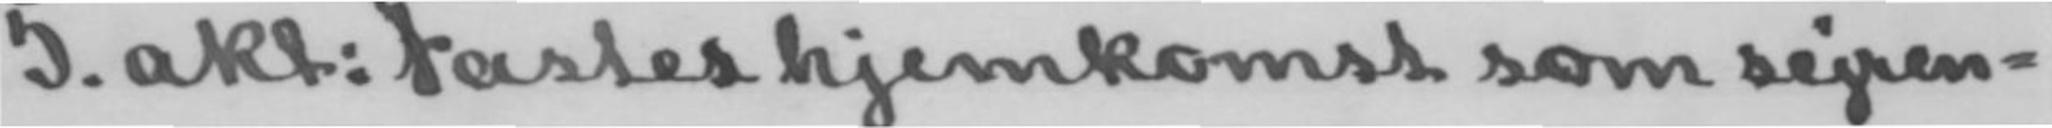5. akt: Fastes hjemkomst som sejren-
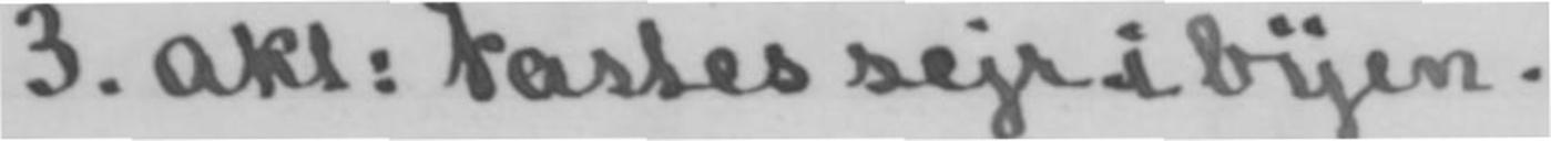3. akt: Fastes sejr .i byen.
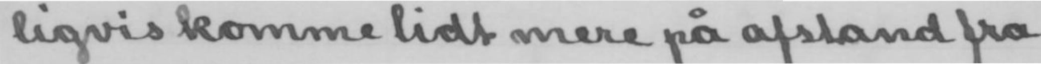ligvis komme lidt mere på afstand fra
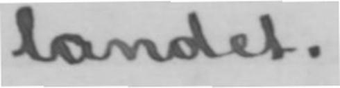landet.
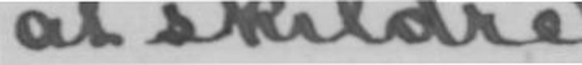at skildre
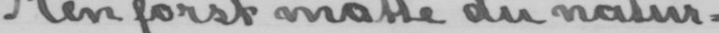Men først måtte du natur-
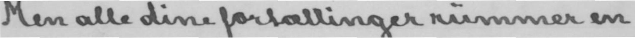Men alle dine fortællinger rummer en
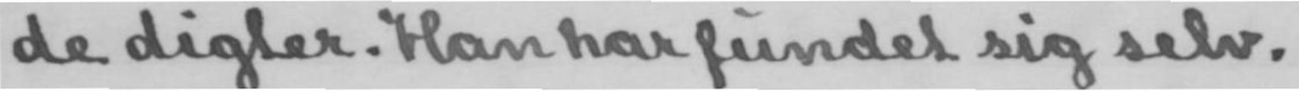de digter. Han har fundet sig selv.
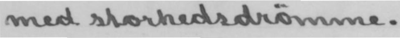med storhedsdrømme.
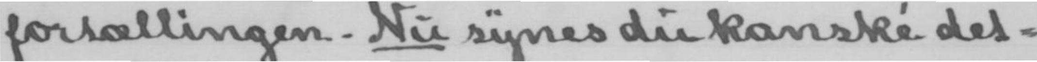fortællingen. Nu synes du kanské det-
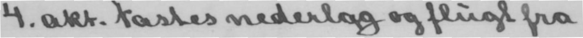4. akt. Fastes nederlag og flugt fra
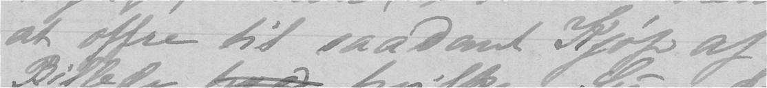at offre til saadant Kjøp af
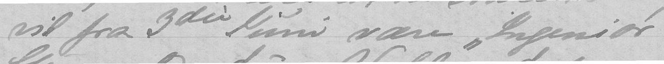vil fra 3die Juni være "Ingeniør
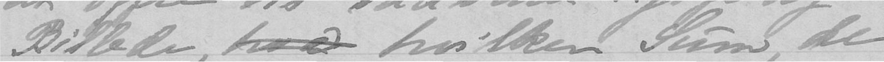Billede, hvad hvilken Sum der
